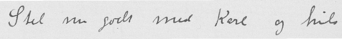Stel nu godt med Karl og hils
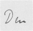Din
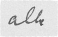alle
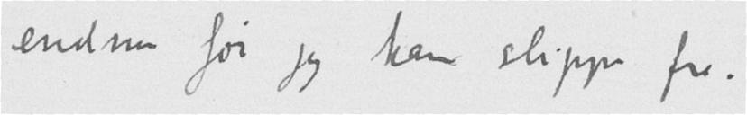endnu før jeg kan slippe fri.
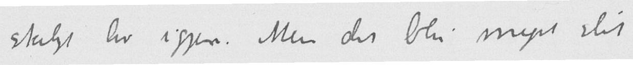skeligt liv igjen. Men det blir meget slit
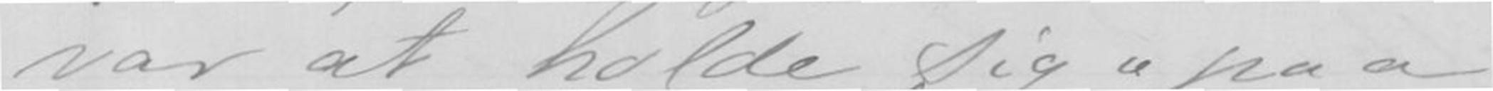var at holde sig paa
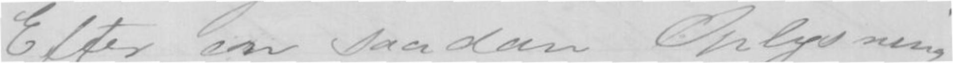Efter en saadan Oplysning
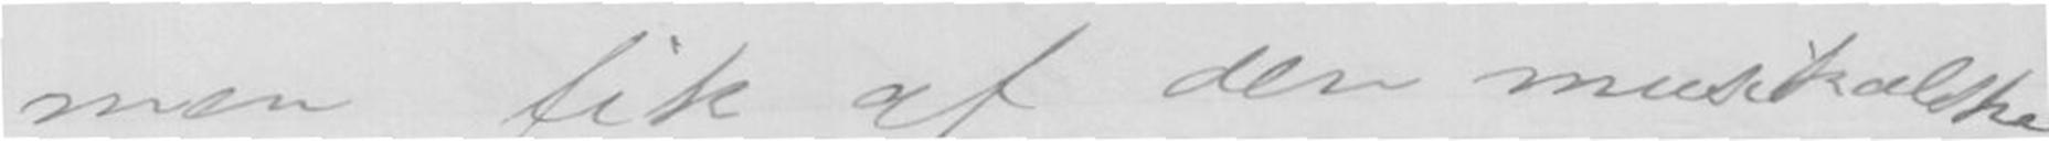men fik af den musikalske
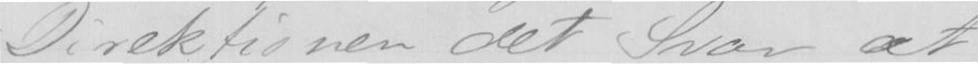Direktionen det Svar at
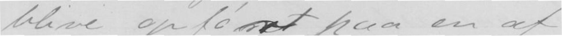blive opført paa en af
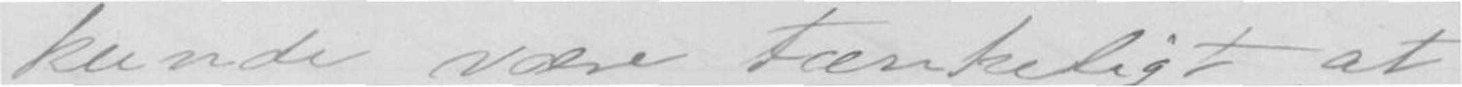kunde være tænkeligt at
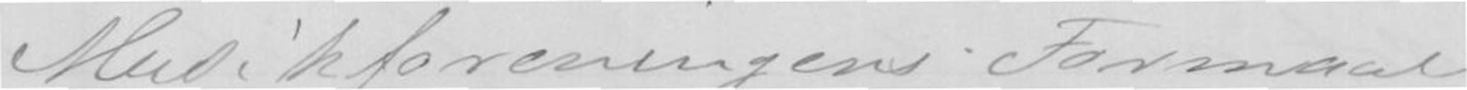Musikforeningens Formaal
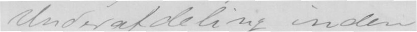Underafdeling inden
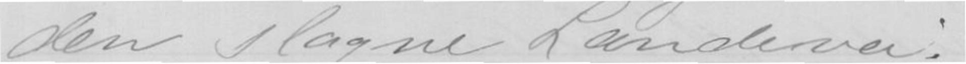den slagne Landevei.
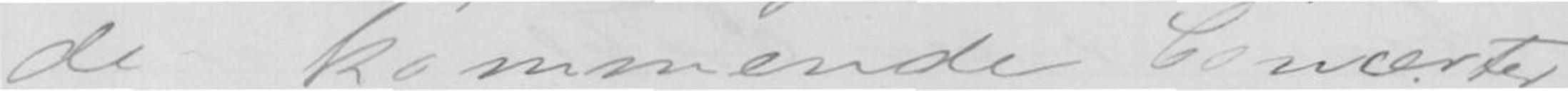de kommende Concerter
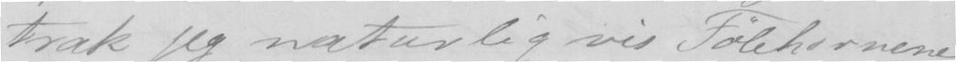trak jeg naturligvis Følehornene
